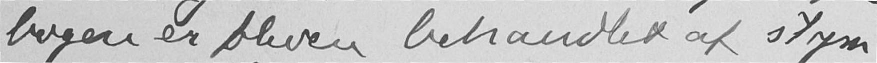bogen er bliven behandlet af stym-
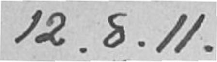12.8.11.
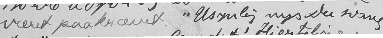været paakrævet. "Usynlig nys Du svang
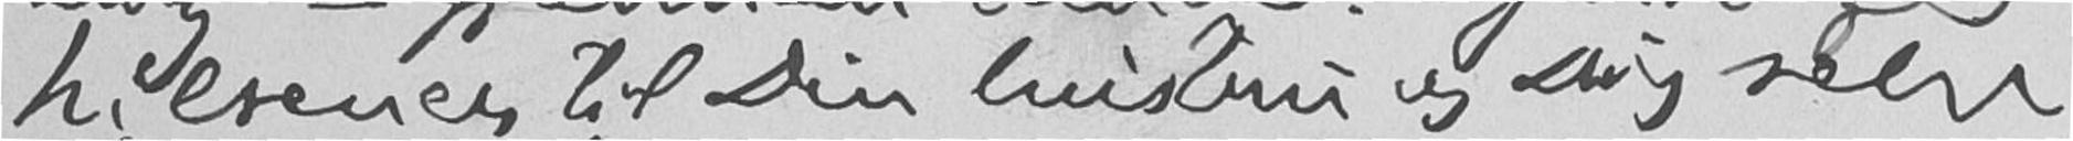hilsener til Din hustru og dig selv
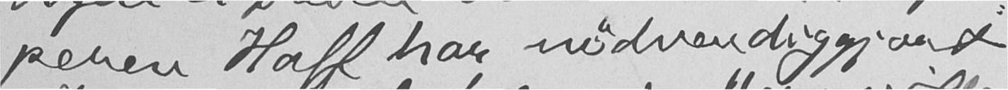peren Hoff har nødvendiggjort
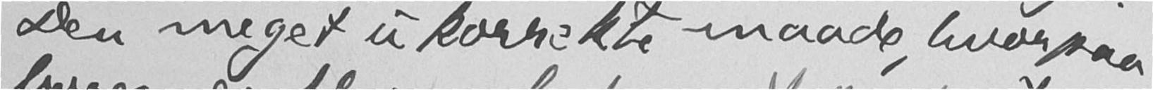Den meget ukorrekte maade, hvorpaa
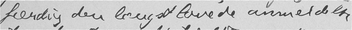færdig den længst lovede anmeldelse
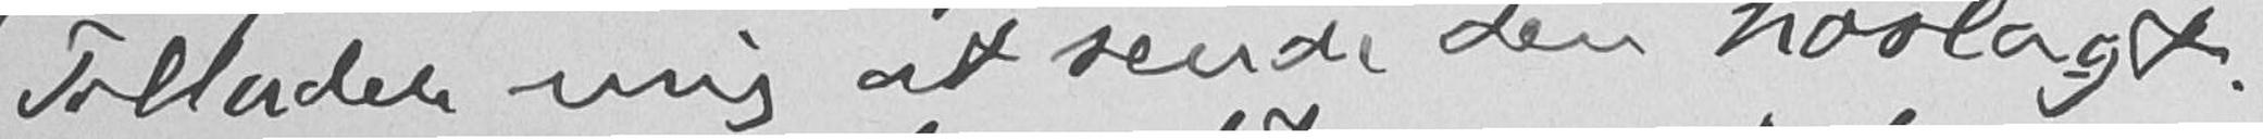Tillader mig at sende den hoslagt.
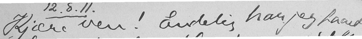Kjære ven! Endelig har jeg faaet
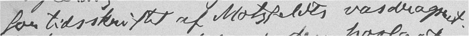for tidsskriftet af Motzfeldts vasdragsret.
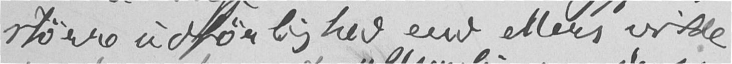større udførlighed end ellers vilde
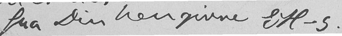fra Din hengivne EH-g.
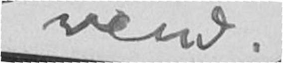vend.
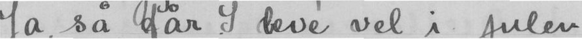Ja, så får I leve vel i julen
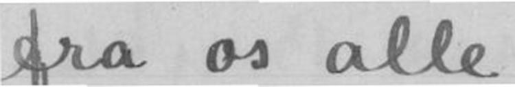fra os alle
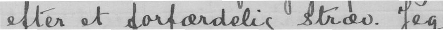efter et forfærdelig stræv. Jeg
